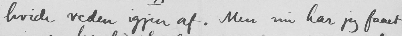hvide veden igjen af. Men nu har jeg faaet
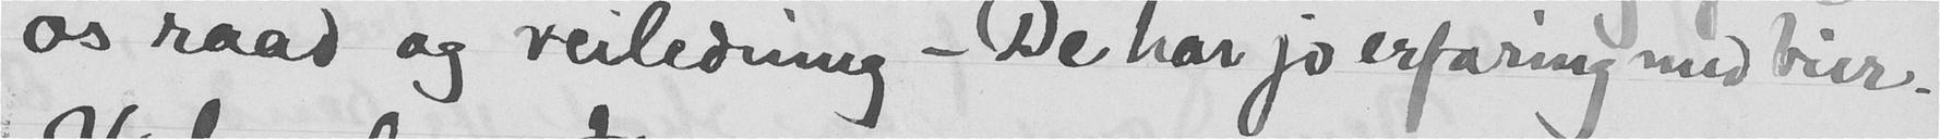os raad og veiledning. - De har jo erfaring med bier.
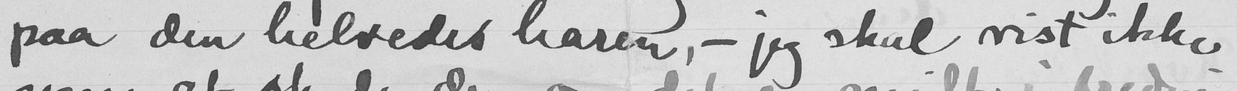paa den helvedes haren, - jeg skal vist ikke
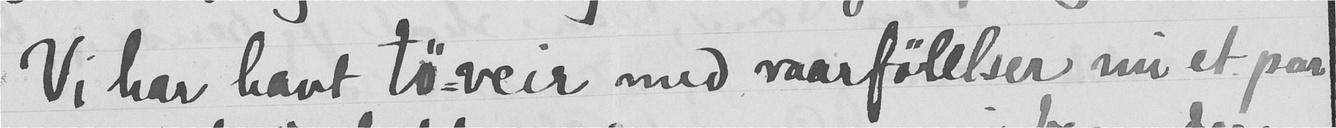Vi har havt tø-veir med vaarfølelser nu et par
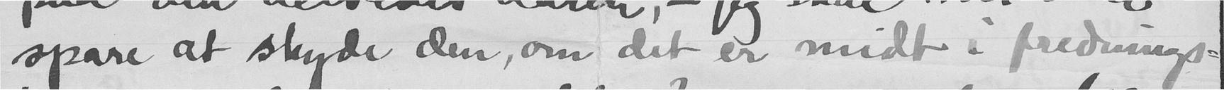spare at skyde den, om det er midt i frednings-
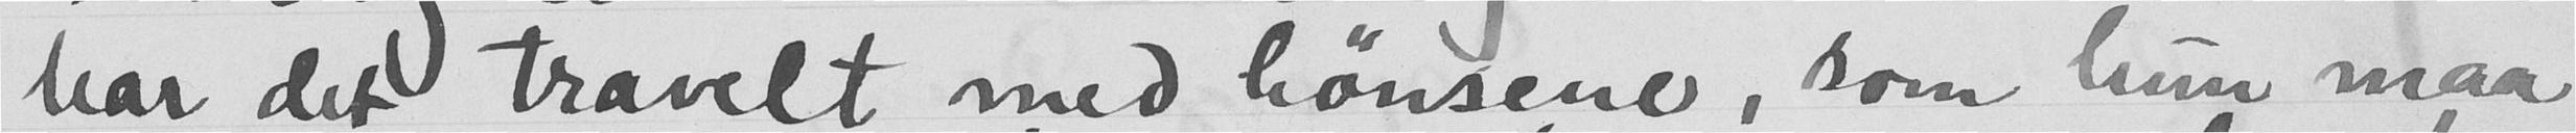har det travelt med hønsene, som hun maa
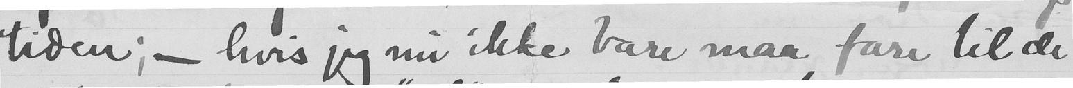tiden; - hvis jeg nu ikke bare maa fare til de
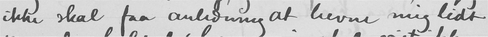ikke skal faa anlednig at hevne mig lidt
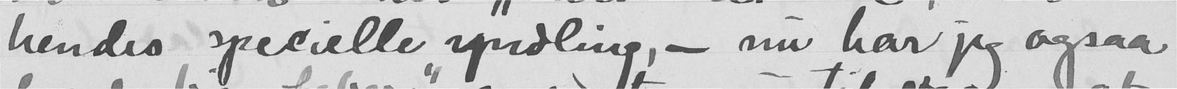hendes specielle yndling, - nu har jeg ogsaa
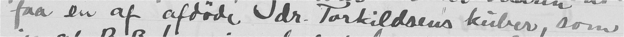faa en af afdøde dr. Torkildsens kuber, som
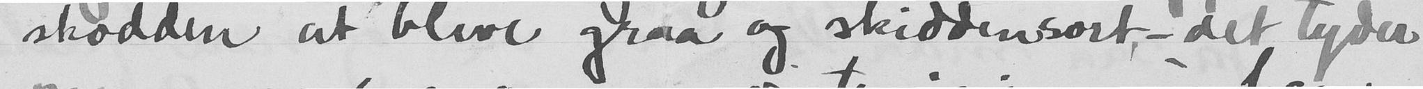skødden at blive graa og skiddensort, - det tyder
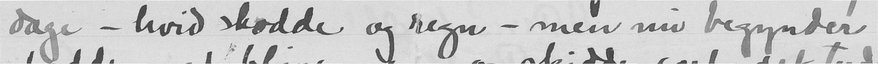dage - hvid skodde og regn - men nu begynder
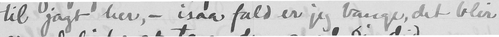til jagt her, - isaa fald er jeg bange, det blir
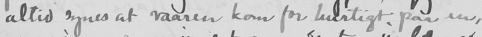altid synes at vaaren kom for hurtigt, paa en,
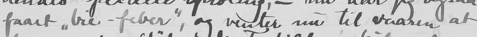faaet "bie-feber", og venter nu til vaaren at
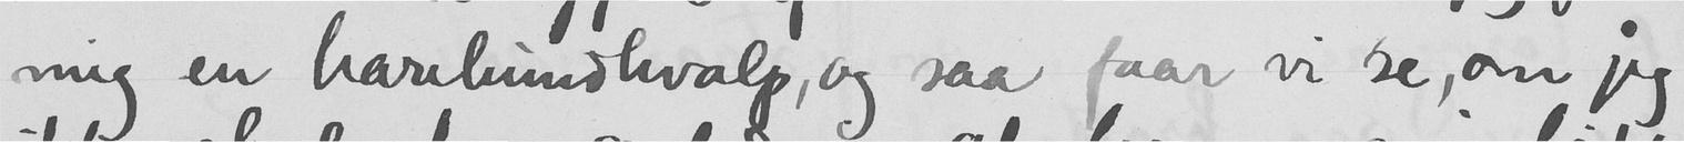mig en harehundhvalp, og saa har vi se, om jeg
